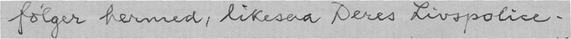følger hermed, likesaa Deres Livspolice.
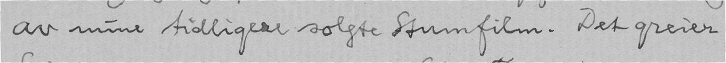av mine tidligere solgte Stumfilm. Det greier
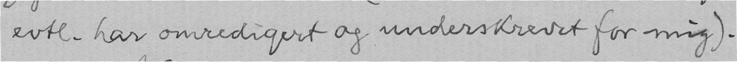evtl. har omredigert og underskrevet for mig).
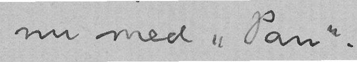nu med "Pan".
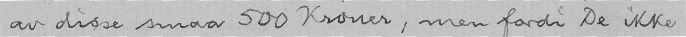av disse smaa 500 Kroner, men fordi De ikke
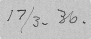17/3. 36.
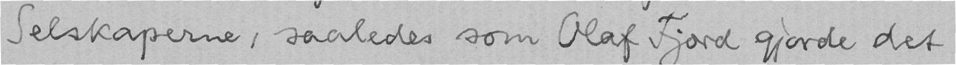Selskaperne, saaledes som Olaf Fjord gjorde det
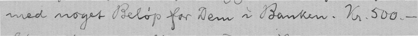med noget Beløp for Dem i Banken. Kr. 500. -
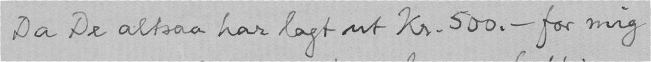Da De altsaa har lagt ut Kr. 500. - for mig
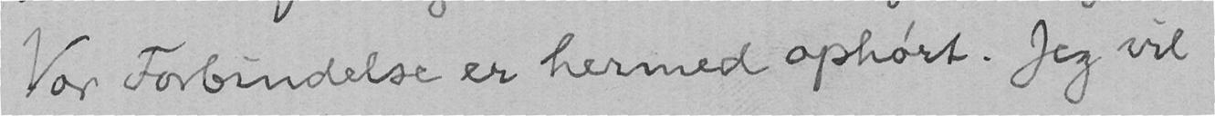Vor Forbindelse er hermed ophørt. Jeg vil
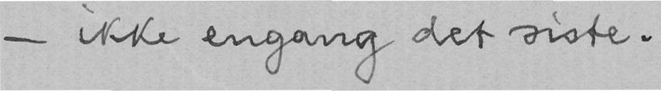- ikke engang det siste.
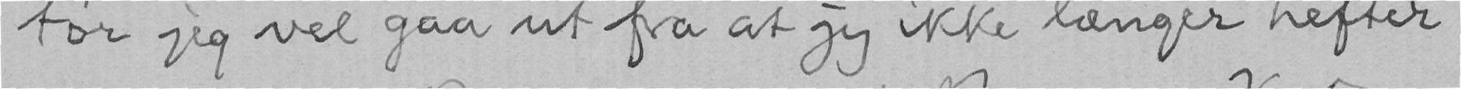tør jeg vel gaa ut fra at jeg ikke længer hefter
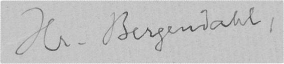Hr. Bergendahl,
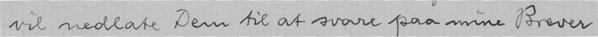vil nedlate Dem til at svare paa mine Brever
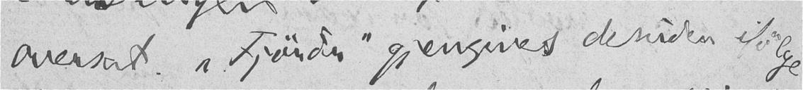oversat. "Fjörðr" gjengives desuden ifølge
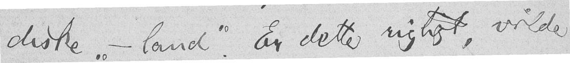diske "-land". Er dette rigtigt, vilde
